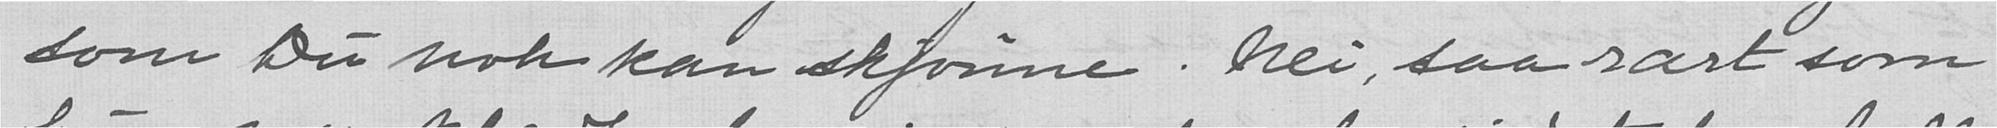som Du nok kan skjønne. Nei, saa rart som
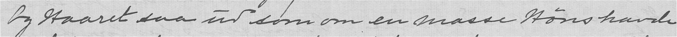Og haaret saa ud som om en masse Høns havde
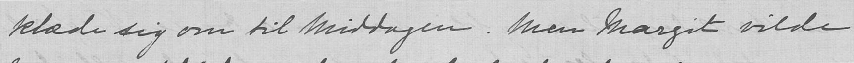klæde sig om til Middagen. Men Margit vilde
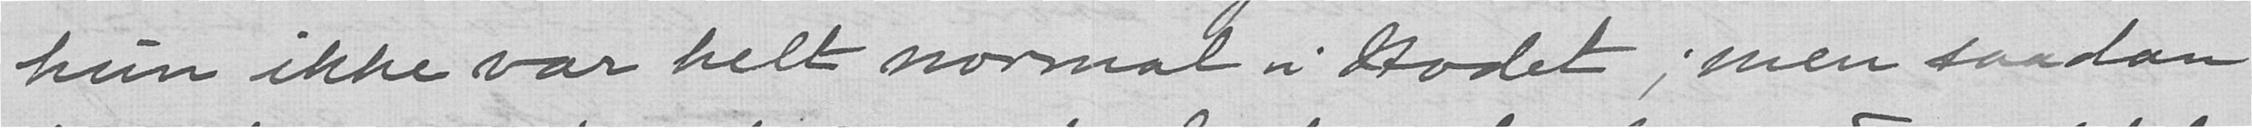hun ikke var helt normal i Hodet; men saadan
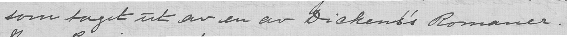som taget ut av en av Dickens's Romaner.
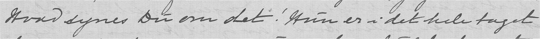Hvad synes Du om det! Hun er i det hele taget
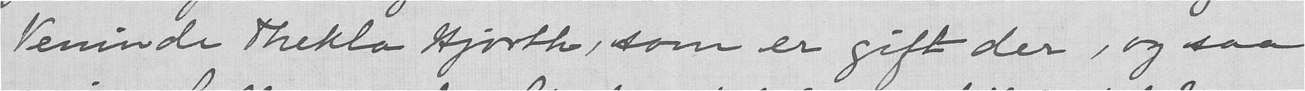Veninde Thekla Hjorth, som er gift der, og saa
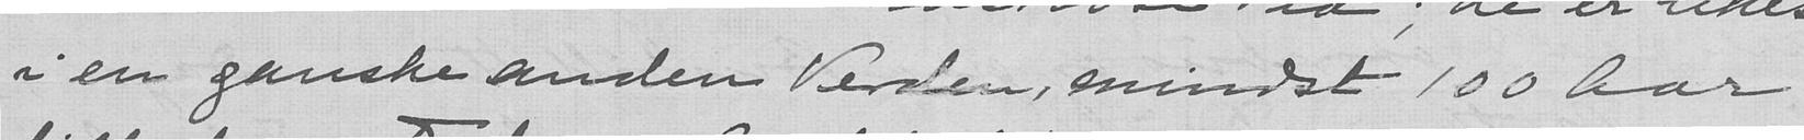i en ganske anden Verden, mindst 100 Aar
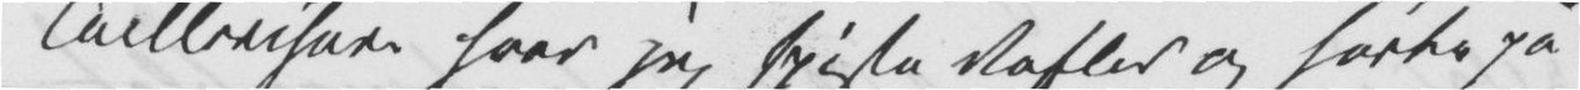Tuillerihave hvor jeg spiste Vafler og hørte på
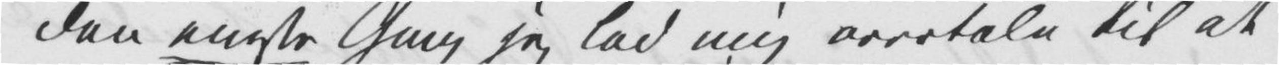den eneste Gang jeg lod mig overtale til at
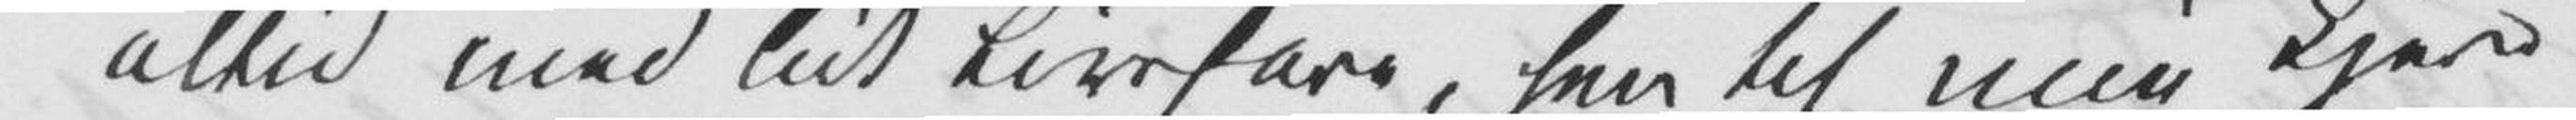altid med lidt Livsfare, hen til min kjere
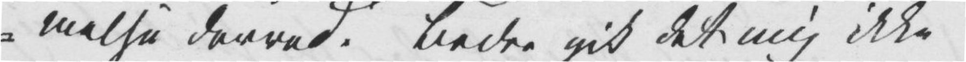melse derved. Bedre gik det mig ikke
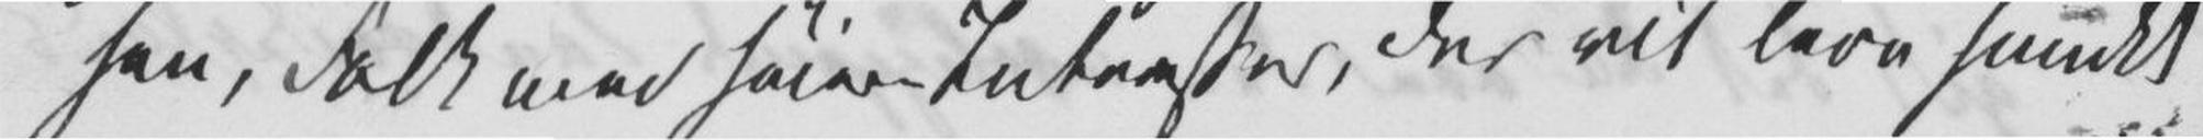hen, Folk med høiere Interesser, der vil leve smukt
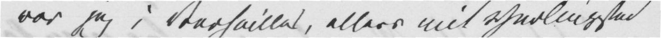var jeg i Versailles, ellers mit Yndlingsted
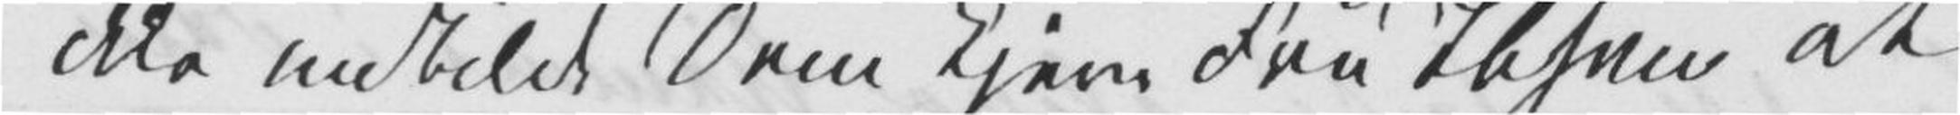ikke indbilde Dem kjere Fru Ibsen at
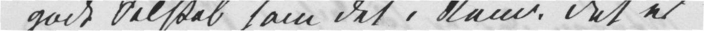godt Selskab som det i Rom. Det er
